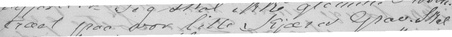træet paa vor lille Kjære Grav. Skal
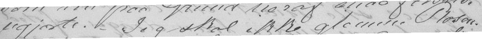ugjorte. Jeg skal ikke glemme Rosen-
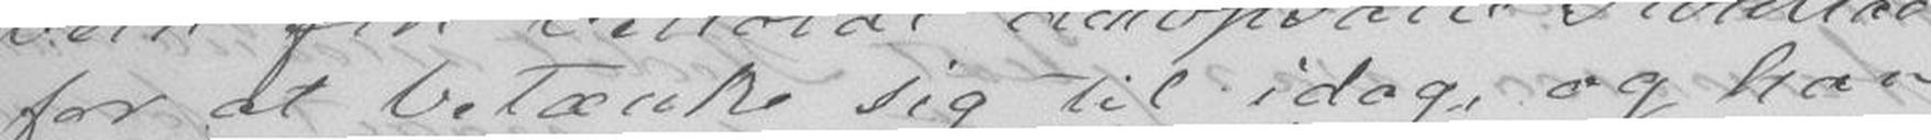for at betænke sig til idag og han
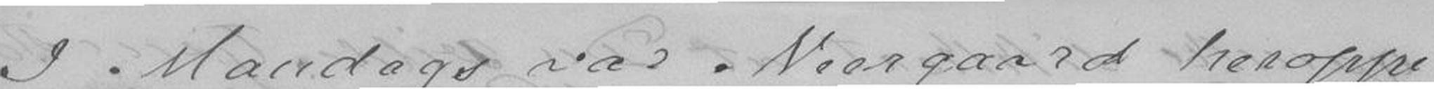I Mandags var Neergaard heroppe
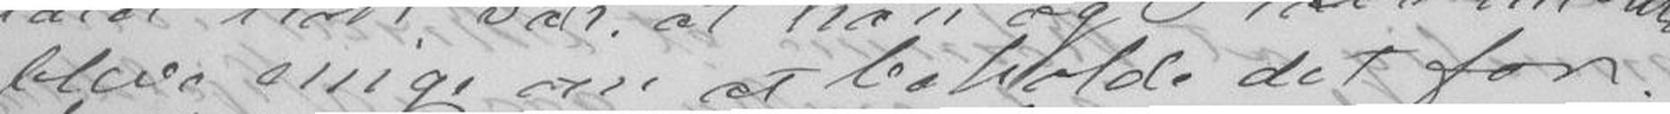bleve enige om at beholde det for
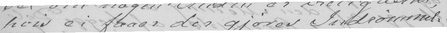hvis ei faaer der gjøres Indrømmel-
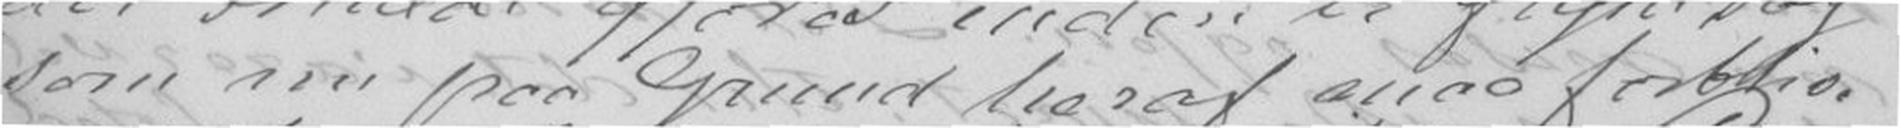som nu paa Grund heraf enno forblive
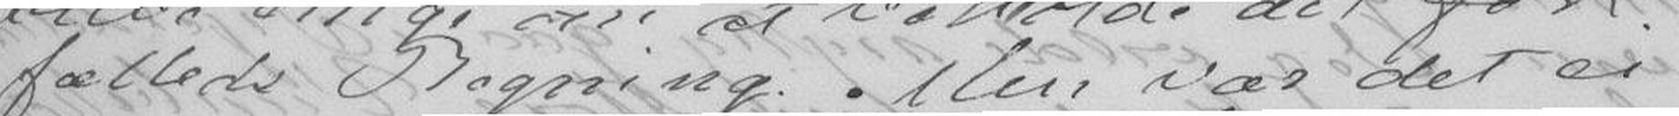fælleds Regning. Men var det ei
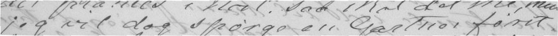jeg vil dog spørge en Gartner først
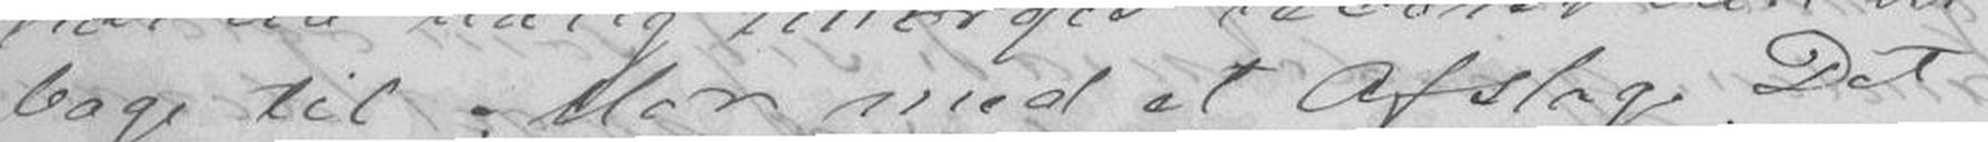bage til Mor med et Afslag. Det
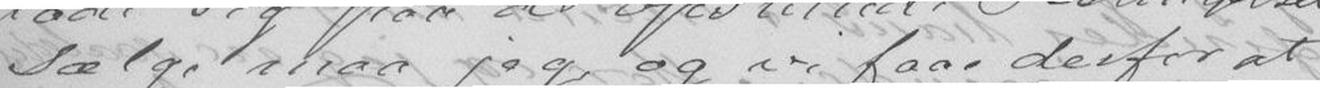Sælge maa jeg og vi faae derfor at
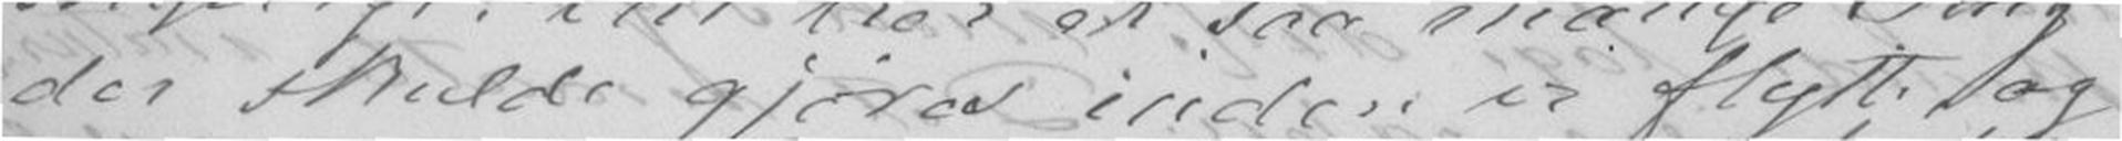der skulde gjøres inden vi flytte, og
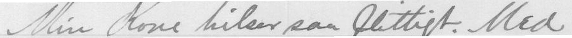Min Kone hilser saa flittigt. Med
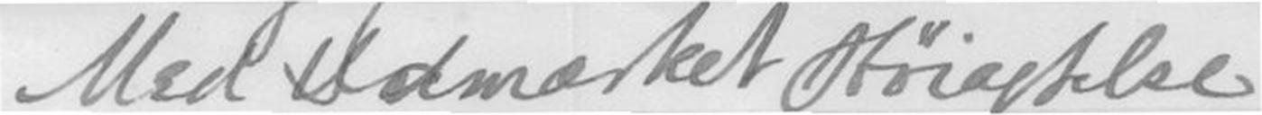Med Udmærket Højagtelse
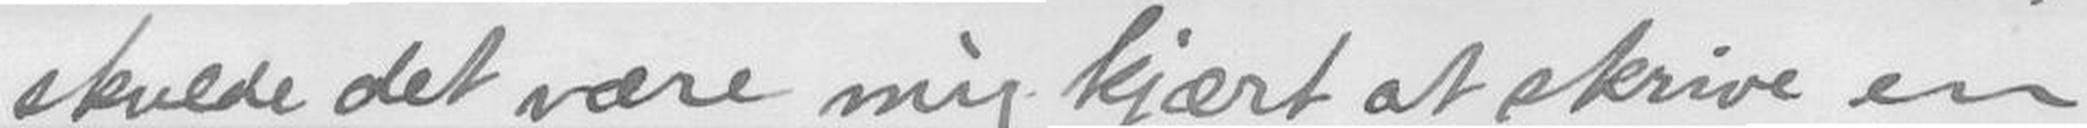skulde det være mig kjært at skrive en
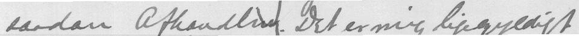saadan Afhandling. Det er mig ligegyldigt
